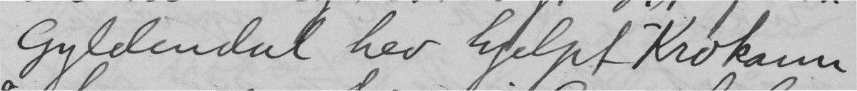Gyldendal hev hjelpt Krokann
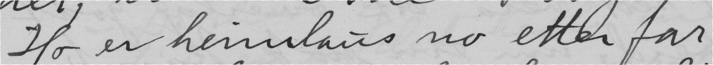Ho er heimlaus no etter far
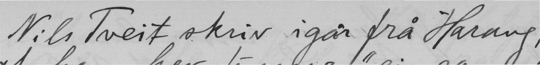Nils Tveit skriv igår fra Harang,
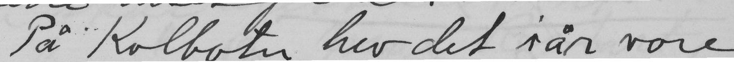På Kolbotn hev det iår vore
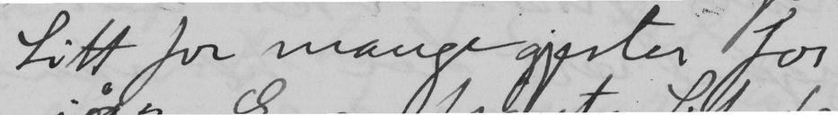Litt for mange gjester for
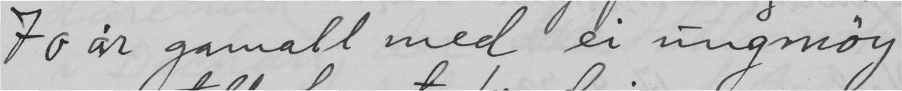70 år gamall med ei ungmöy
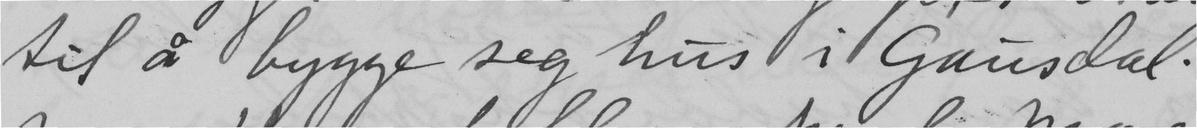til å bygge seg hus i Gausdal.
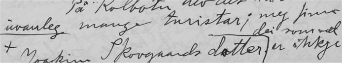uvanleg mange turistar; meg finner dei som væl er ikkje
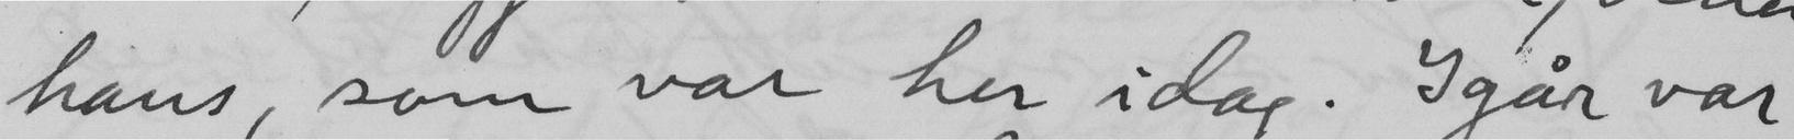hans, som var her idag. Igår var
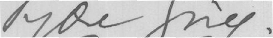Kjære Grieg.
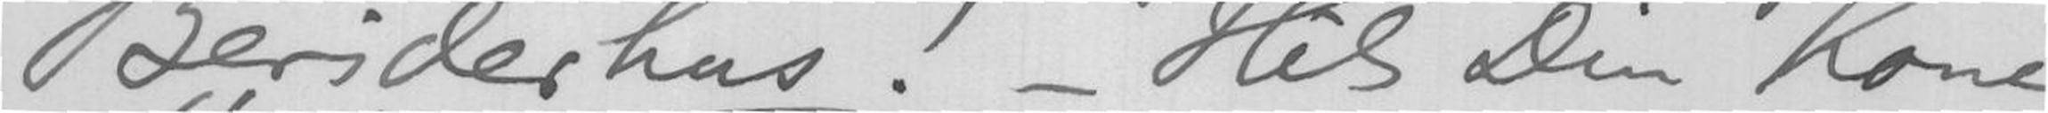Beriderhus! - Hils Din Kone.
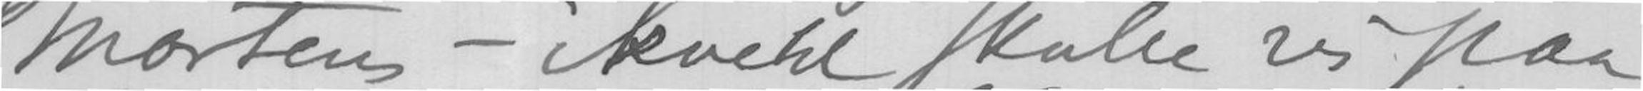Mortens - ikveld skulle vi paa
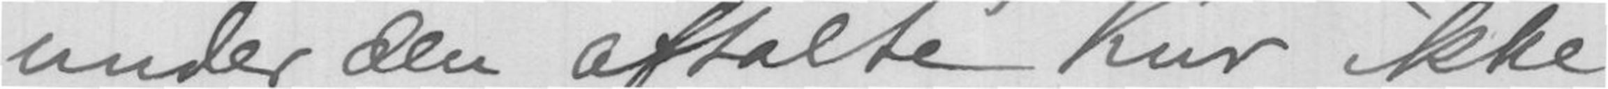under den aftalte Kur ikke
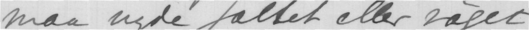maa nyde saltet eller røget
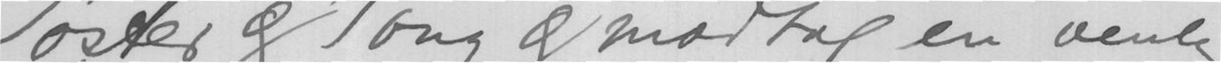Søster og Tony og modtag en venlig
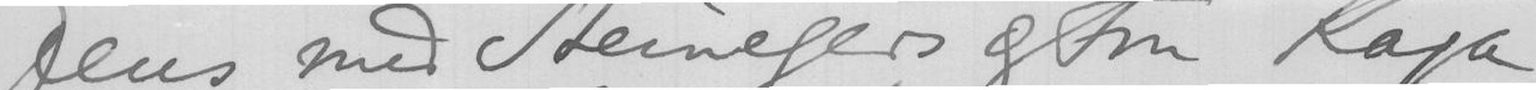Jens med Steinegers og Fru Kaja
